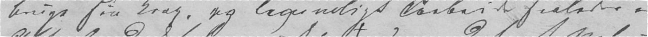bruge sin Krog, og legemligt Arbeide saaledes er
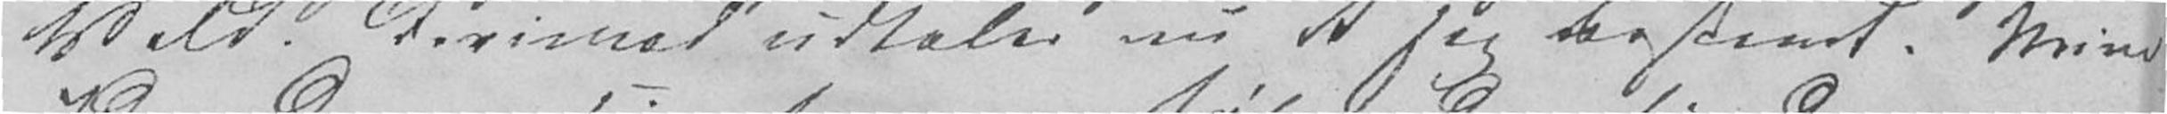Vold. Derimod udtaler nu A sig bestemt. Mine
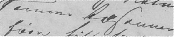samme Ræsonne-
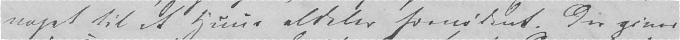noget til et Huus aldeles fornødent. Der gives
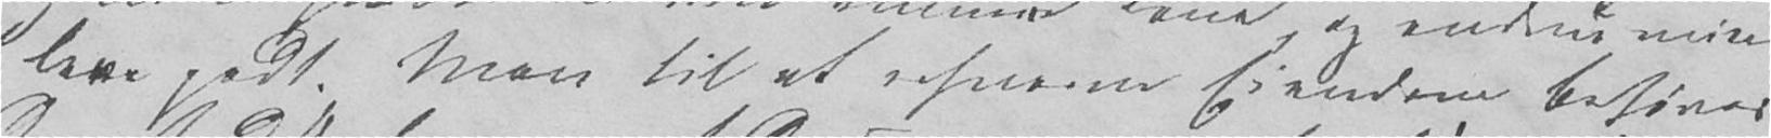leve godt. Men til at erhverve Eiendom behøves
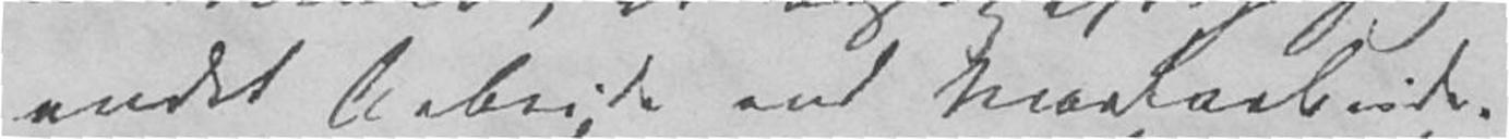andet Arbeide end Maalarbeide.
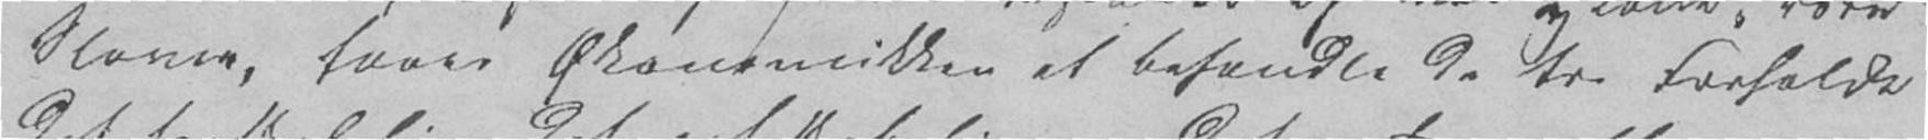Slaver, faaer Økonomikken at behandle de tre Forholde
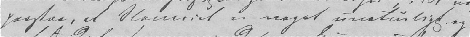paastaa, at Slaveriet er noget unaturligt og
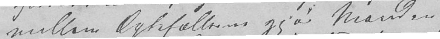mellem Ægtefællene gjør Manden
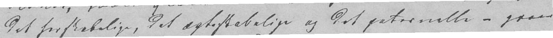det herskabelige, det ægteskabelige og det paternelle – gaaer
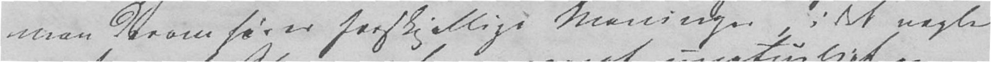man derom hører forskjellige Meninger, i det nogle
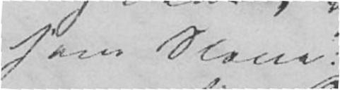som Slave.
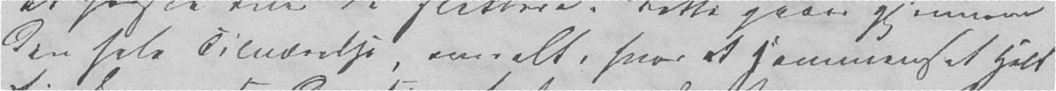den hele Tilværelse, overalt, hvor et sammensat Helt
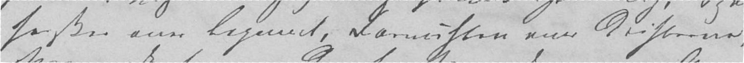hersker over Legemet, Fornuften over Drifterne,
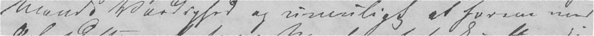Mands Værdighed og umuligt at forene med
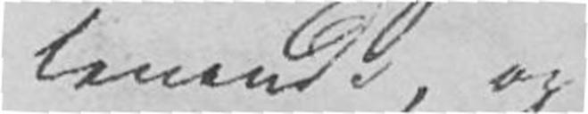levende, og
